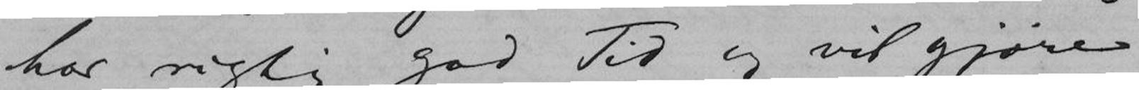har rigtig god Tid og vil gjøre
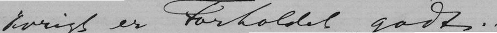øvrigt er Forholdet godt. -
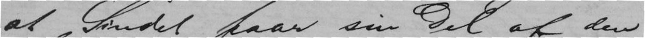at Sindet faar sin Del af den
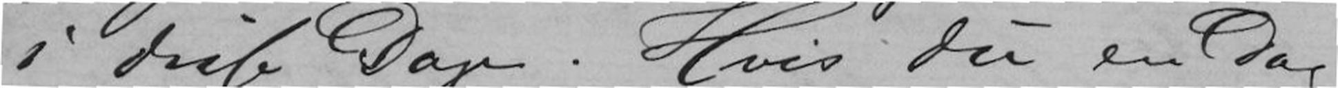i disse Dage. Hvis du en Dag
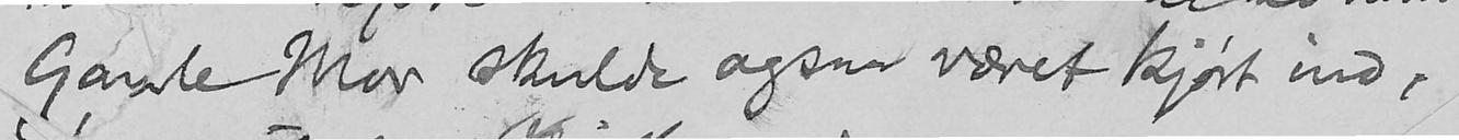Gamle Mor skulde ogsaa været kjørt ind,
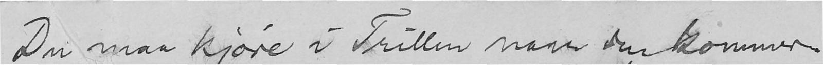Du maa kjøre i Trillen naar den kommer.
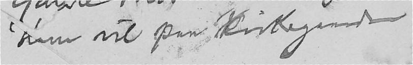hun vil paa Kirkegaarden
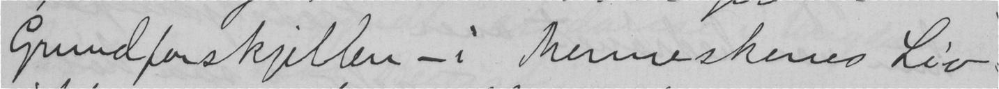Grundforskjellen - i Menneskenes Liv
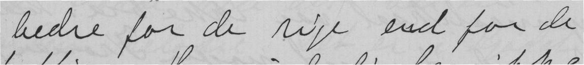bedre for de rige end for de
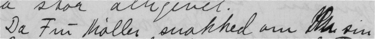Da Fru Møller snakked om  sin
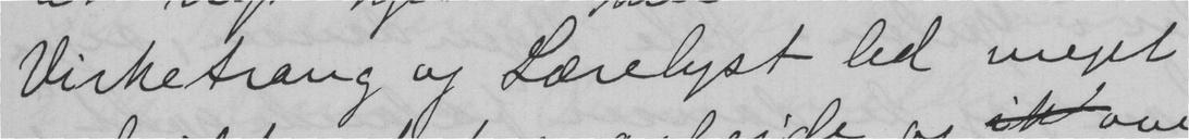Virketrang og Lærelyst led meget
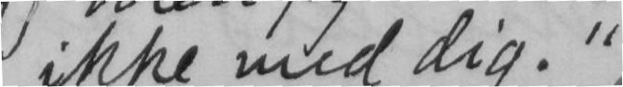ikke med dig."
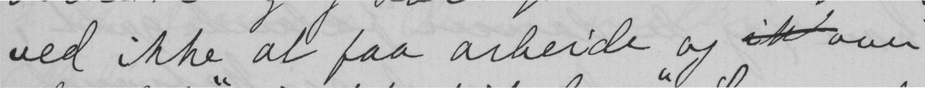ved ikke at faa arbeide og  over
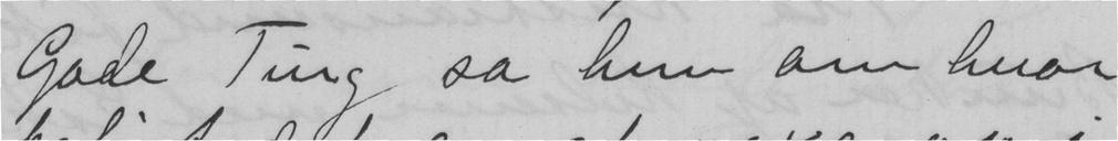Gode Ting sa hun om hvor
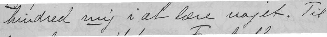hindred mig i at lære noget. Til
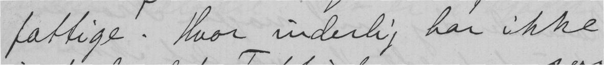fattige. Hvor inderlig har ikke
